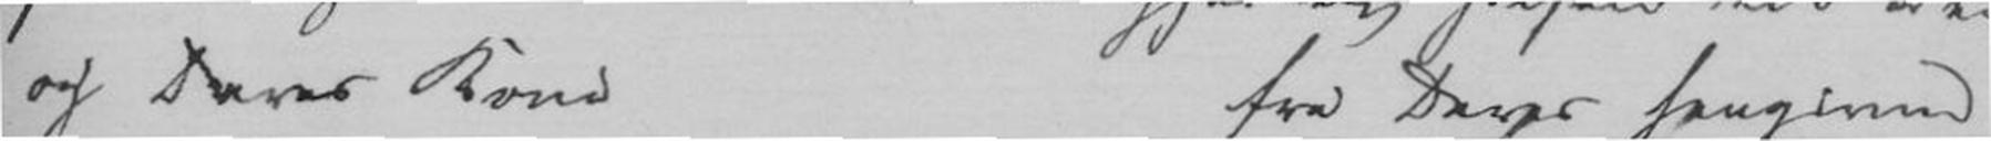og Deres Kone fra Deres hengivne
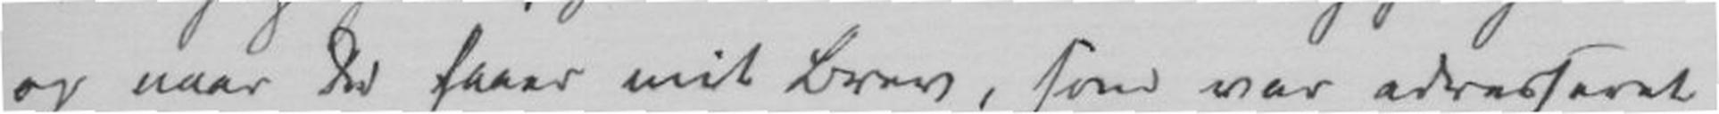og naar De faaer mit Brev, som var adresseret
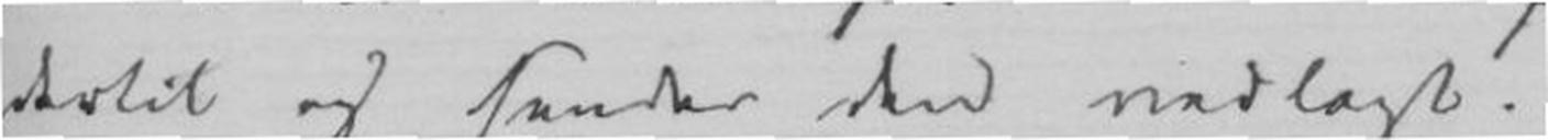dertil og denser den vedlagt.
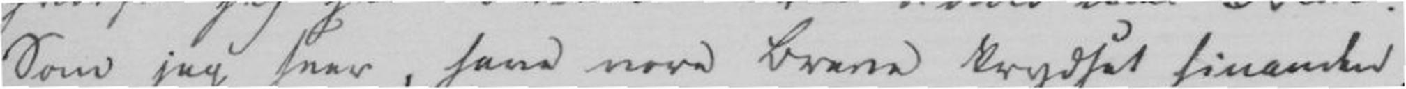Som jeg seer, have vore Breve krydset hinnanden,
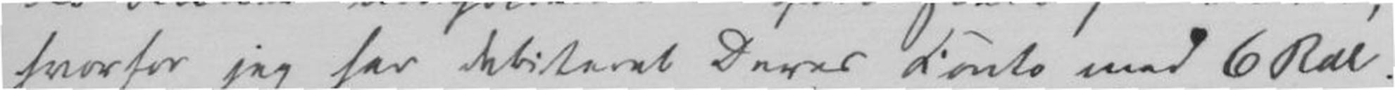hvorfor jeg har debiteret Deres Konto med 6 Rdl.
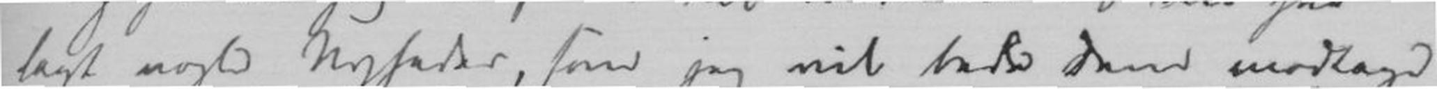lagt nogle Nyheder, som jeg vil bede Dem modtage,
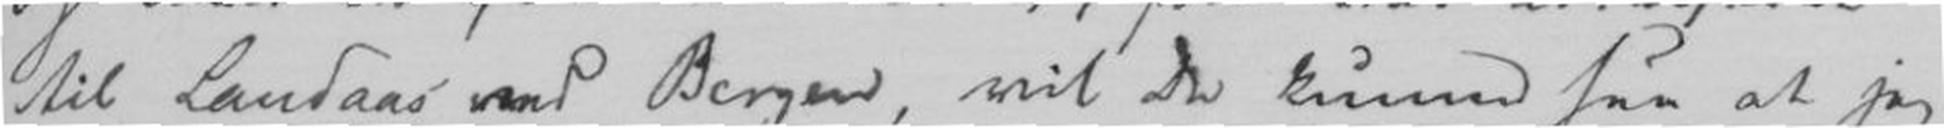til Landaas ved Bergen, vil De kunne see at jeg
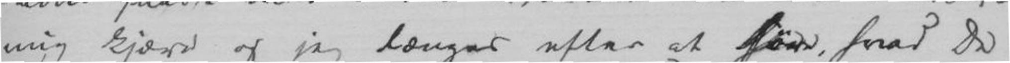mig kjære og jeg længes efter at høre, hvad De
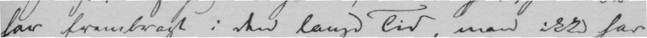gar frembragt i den lange Tid, men ikke har
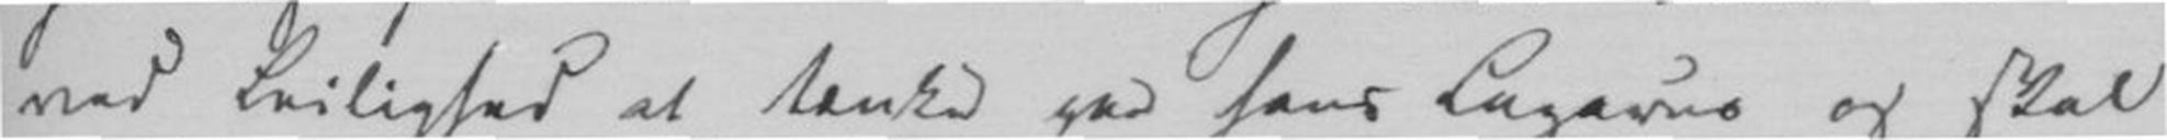ved Leilighed at tænke paa hans Lazarus og skal
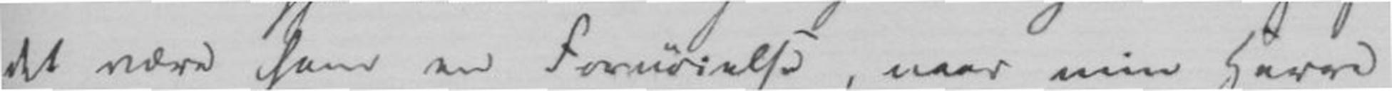det være som en Fornøielse, naar min Herre
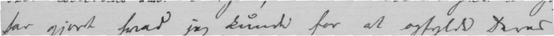har gjort hvad jeg kunde for at opfylde Deres
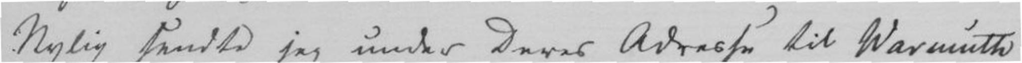Nylig sendte jeg under Deres Adresse til Warmuth
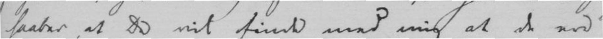haager, at De vil finde med mig at de ere
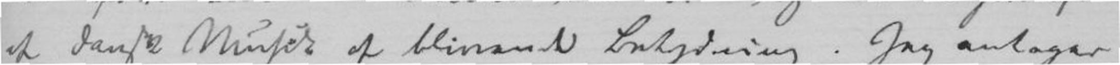af dansk Musik af blivende Belysning. Jeg antager
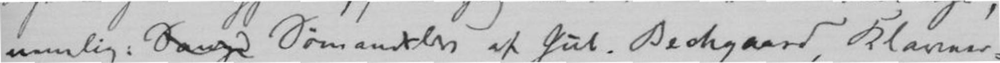nemlig:Sange Sømanden af Jul. Bechgaard, Klaver-
